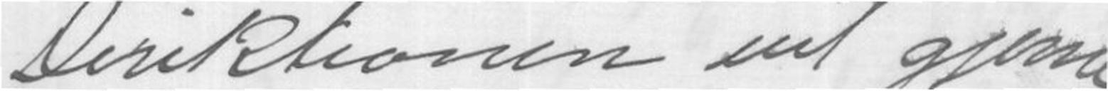Direktionen vil gjerne
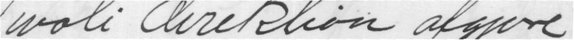tivoli direktion afgjøre
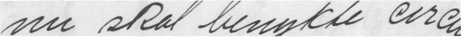nu skal benytte circus
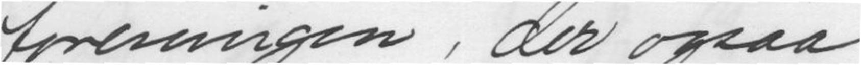foreningen, der ogsaa
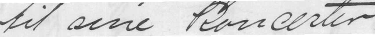til sine koncerter.
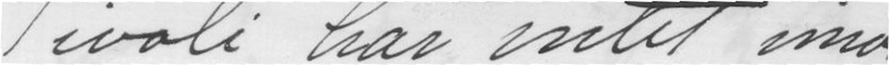Tivoli har intet imod
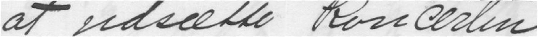at udsætte koncerten
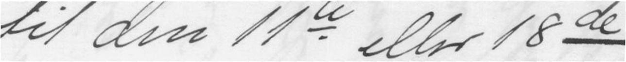til den 11te eller 18de.
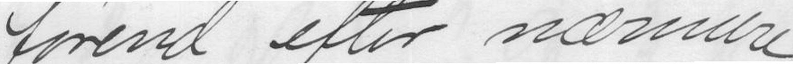førend efter nærmere
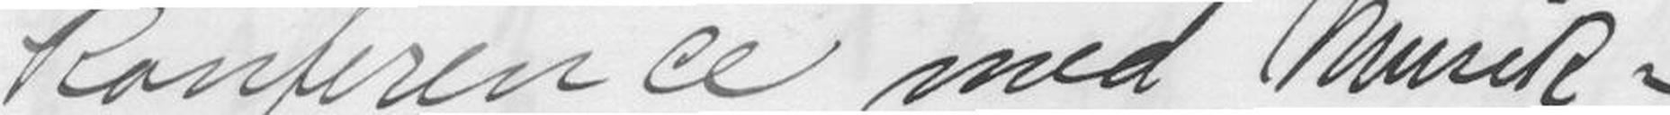konference med Musik-
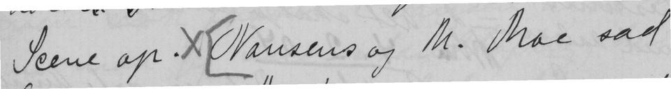Scene op. Nansens og M. Moe sad
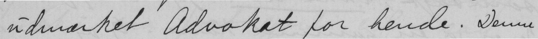udmærket Advokat for hende. Denne
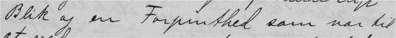Blik og en Forpinthed som var til
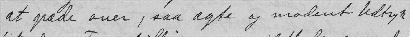at græde over, saa ægte og modent Udtryk
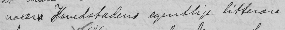voxer Hovedstadens egentlige litterære
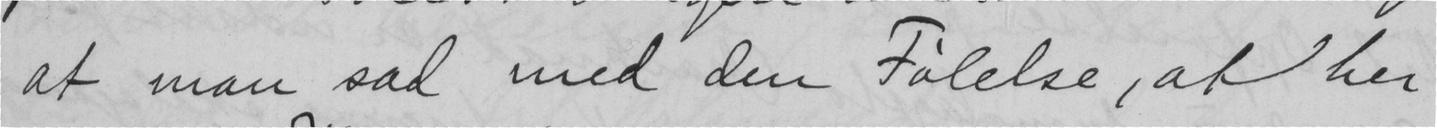at man sad med den Følelse, at her
